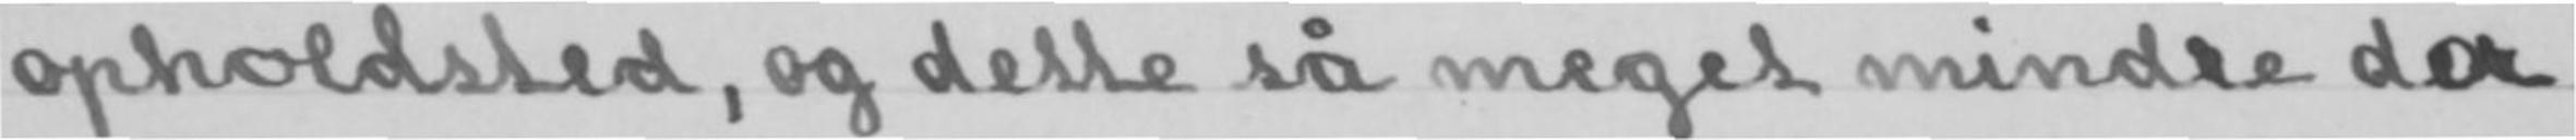opholdsted, og dette så meget mindre da
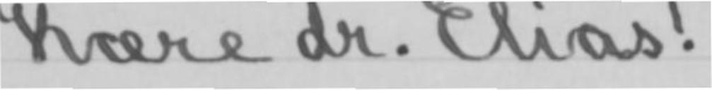Kære dr. Elias!
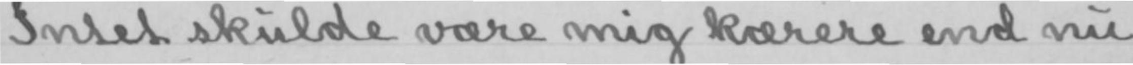Intet skulde være mig kærere end nu
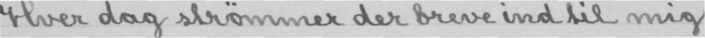Hver dag strømmer der breve ind til mig
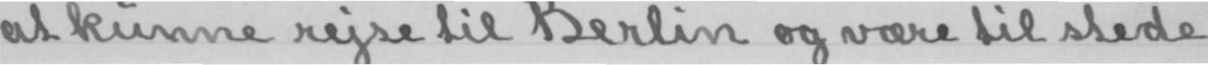at kunne rejse til Berlin og være til stede
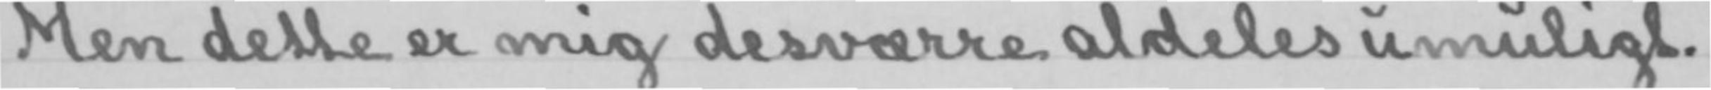Men dette er mig desværre aldeles umuligt.
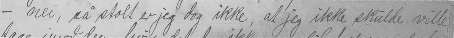- nei, så stolt er jeg dog ikke, at jeg ikke skulde ville
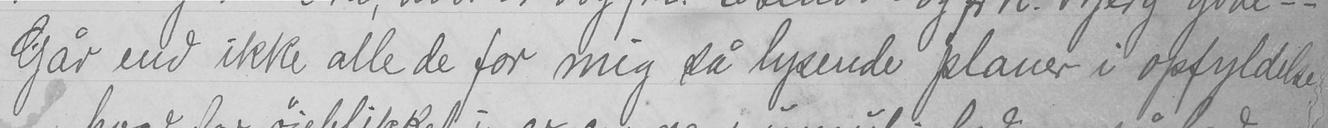Går end ikke alle de for mig så lysende planer i opfyldelse
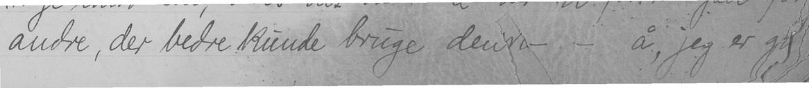andre, der bedre kunde bruge den - - å, jeg er g
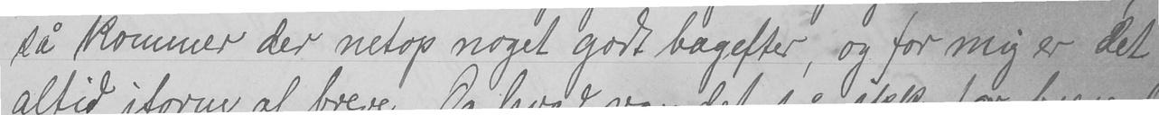så kommer der netop noget godt bagefter, og for mig er det
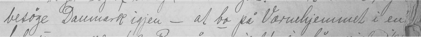besøge Danmark igjen - at bo på Værnehjemmet i en
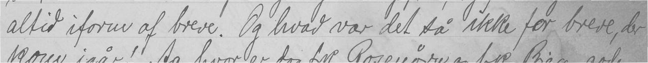altid iform af breve. Og hvad var det så ikke for breve, der
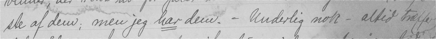ste af dem; men jeg har dem. - Underlig nok - altid træffer
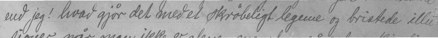end jeg! hvad gjør det med et skrøbeligt legeme og bristede illu-
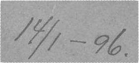14/1-96.
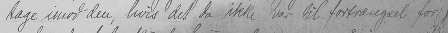tage imod den, hvis det da ikke var til fortrængsel for
